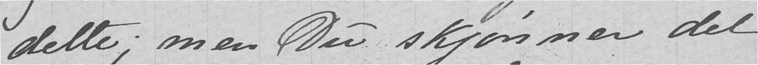dette; men Du skjønner det
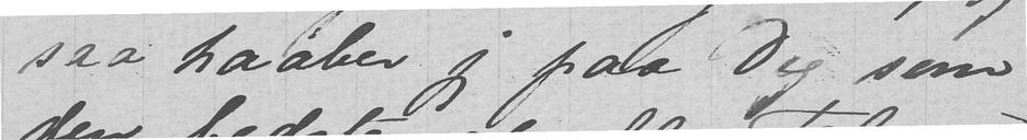saa haaber jeg paa Dig, som
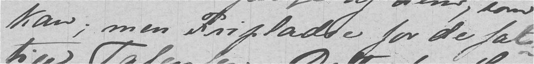kan, men Fripladse for de fat-
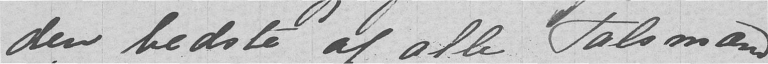den bedste af alle Talsmænd.
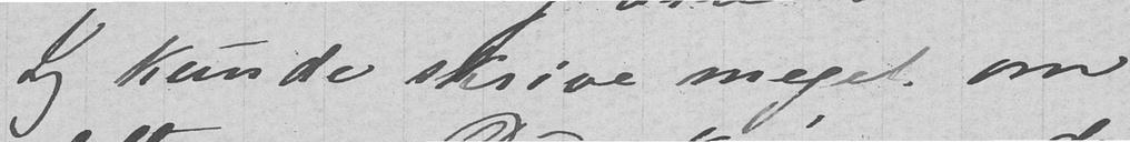Jeg kunde skrive meget om
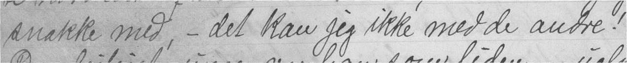snakke med, - det kan jeg ikke med de andre!
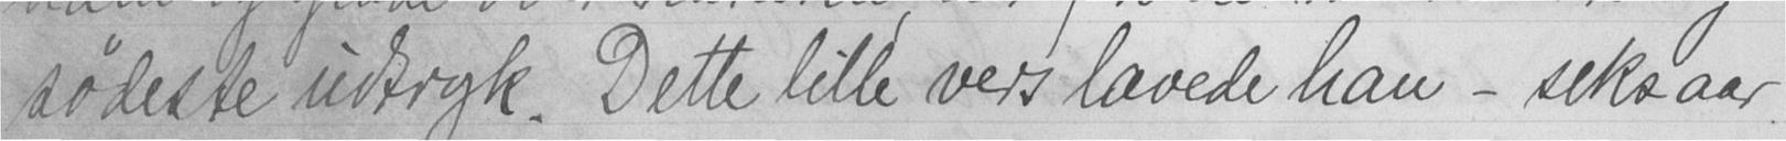sødeste udtryk. Dette lille vers lavede han - seks aar.
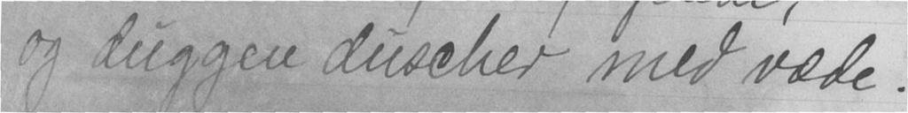og duggen duscher med væde.
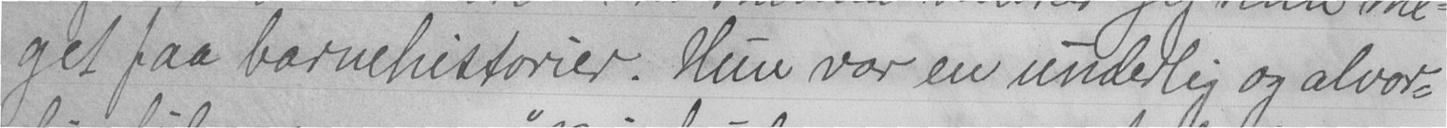get faa barnehistorier. Hun var en underlig og alvor-
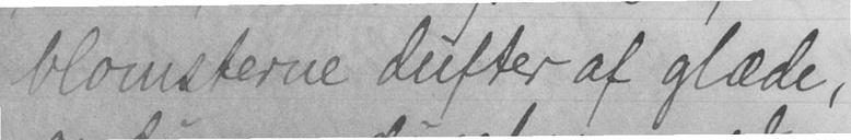blomsterne dufter af glæde,
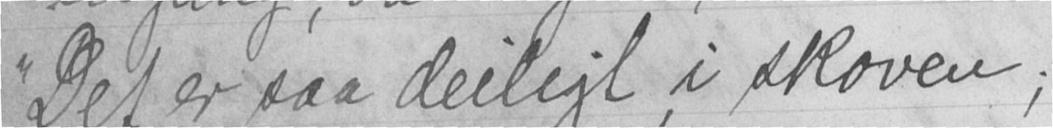"Det er saa deiligt i skoven;
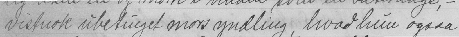vistnok ubetinget mors yndling, hvad hun ogsaa
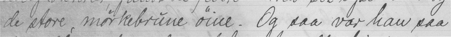de store, mørkebrune øine. Og saa var han saa
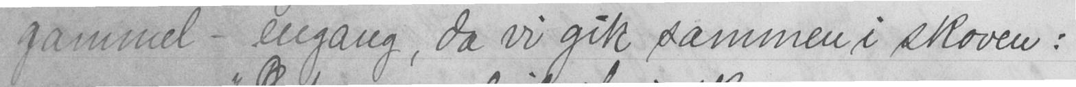gammel - engang, da vi gik sammen i skoven:
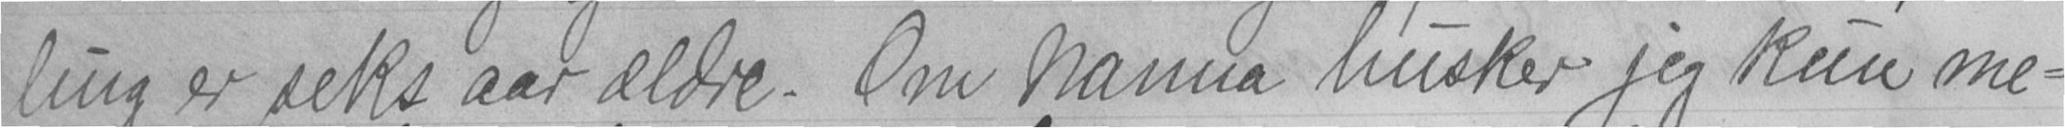ling er seks aar ældre. Om Nanna husker jeg kun me-
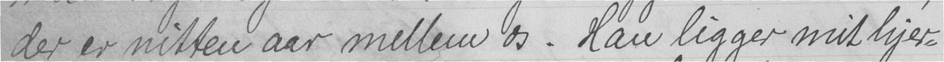der er nitten aar mellem os. Han ligger mit hjer-
